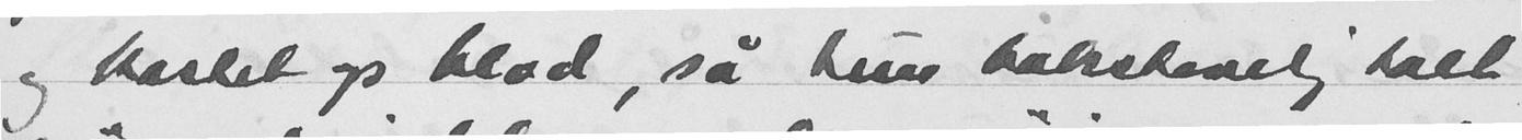og hostet op blod, så hun bokstavelig talt
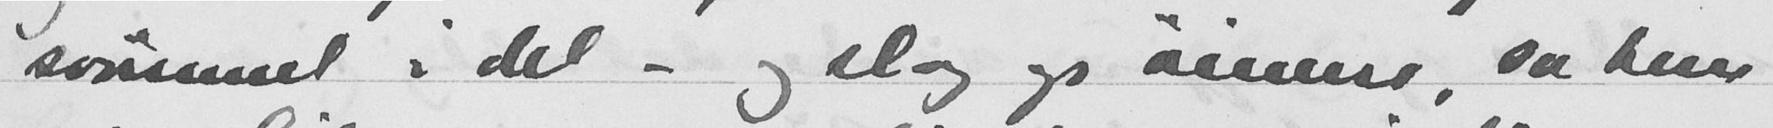svømmet i det - og slog opp øinene, sa hun
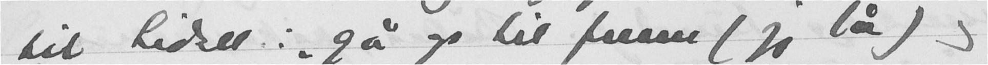til tider: "gå op til feeen (jeg lå) og
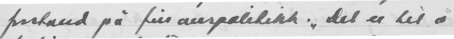forstand på finanspolitikk. "Det er til å
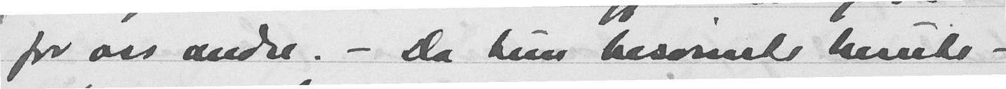for oss andre. - Da hun besvimte herute -
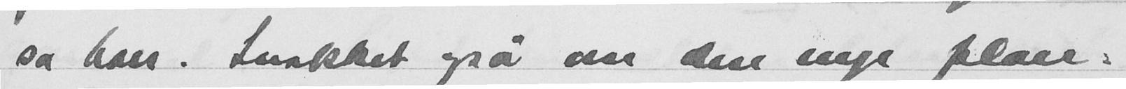sa han. Snakket også om den nye plan-
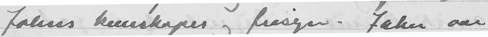Johans kunnskaper og frisyn Johan var
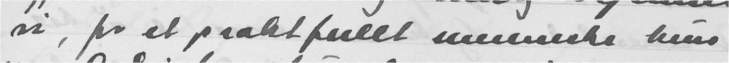vi, for et praktfullt menneske hun
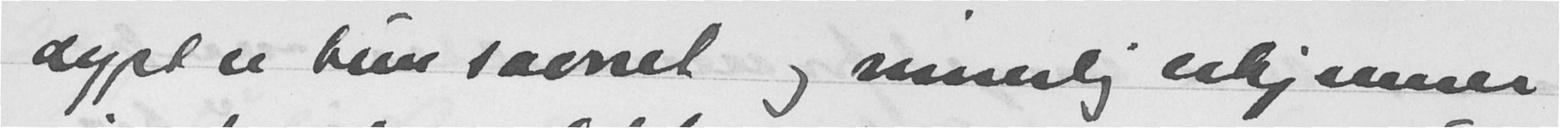dypt er hun savnet og minnelig erkjenner
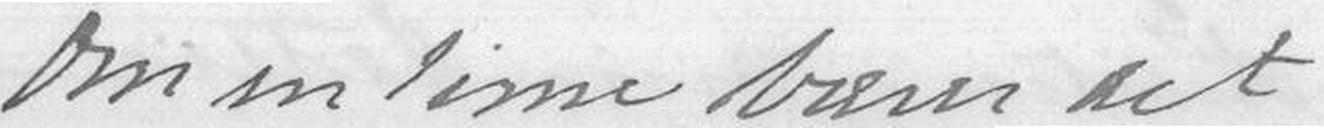Om en Time bærer det
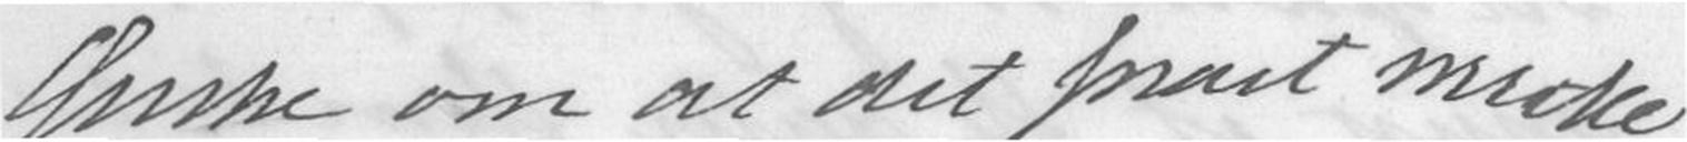Ønske om at det snart maske
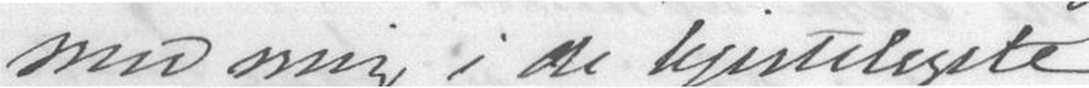med mig i de hjerteligste
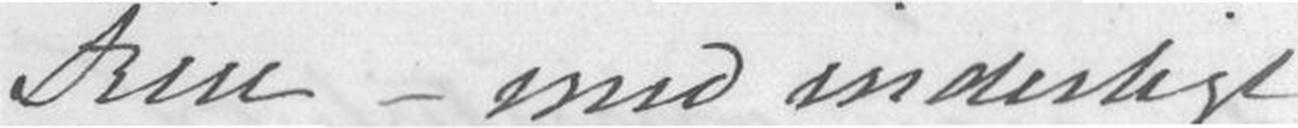Frue - med inderligt
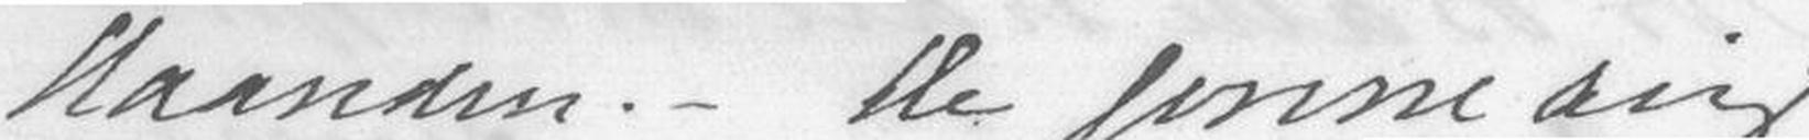Haanden. - De senne dig
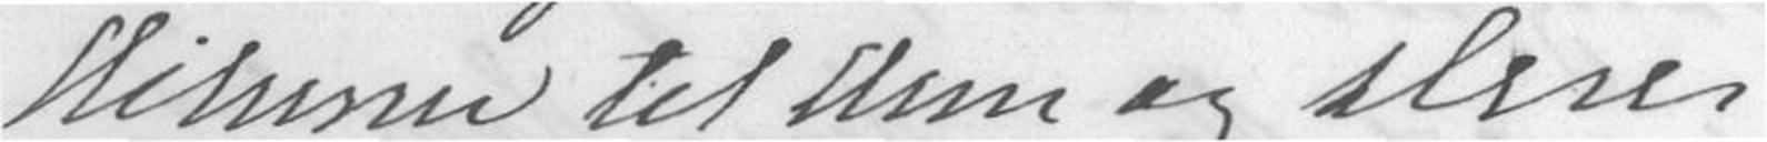Hilsener til Dem og Deres
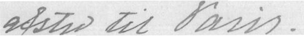afsted til Paris.
